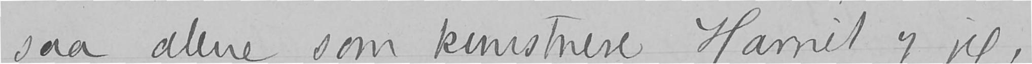saa alene som kunstnere Harriet og jeg,
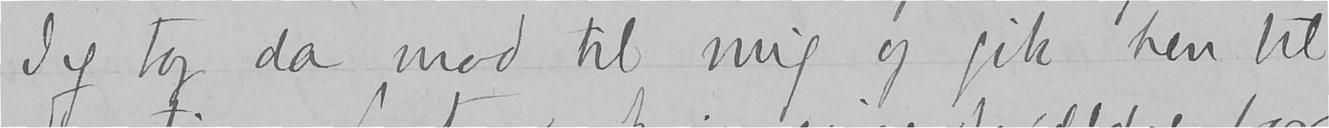Jeg tog da mod til mig og gik hen til
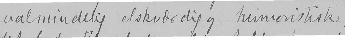ualmindelig elskværdig og humoristisk,
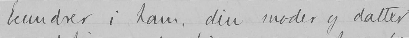beundrer i ham, din moder og datter
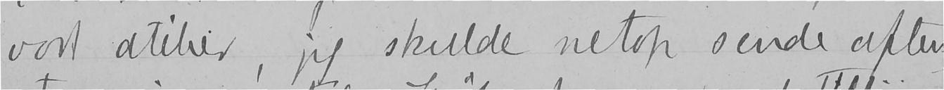vort atélier, jeg skulde netop sende aften
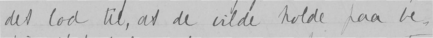det lod til, at de vilde holde paa be-
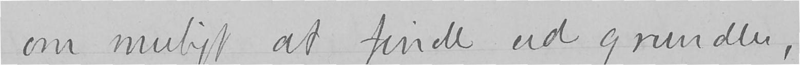om muligt at finde ud grunden,
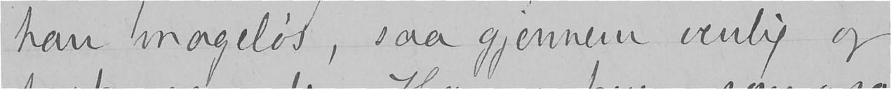han mageløs, saa gjennem venlig og
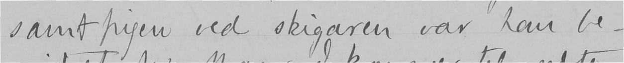samt pigen ved skigaren var han be-
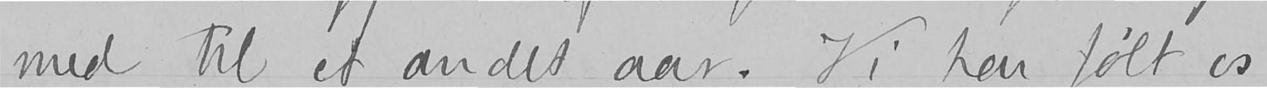med til et andet aar. Vi har følt os
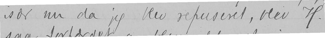især nu da jeg blev refuseret, blev H.
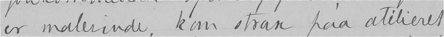er malerinde, kom strax paa atélieret,
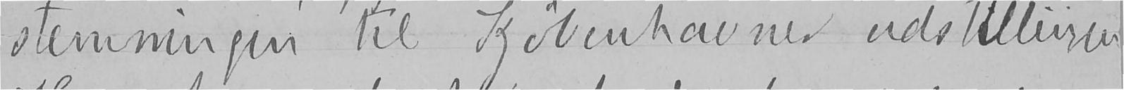stemningen til Kjøbenhavner udstillingen
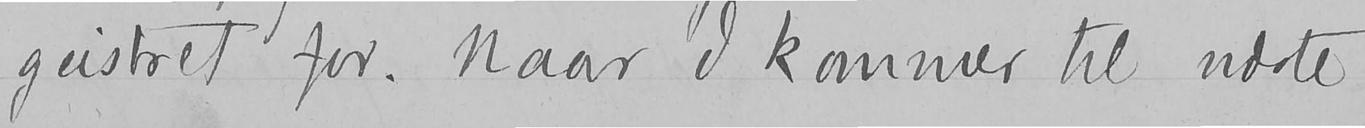geistret for. Naar I kommer til næste
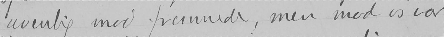uvenlig mod fremmede, men mod os var
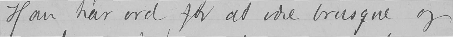Han har ord for at være brusque og
